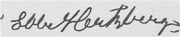Ebbe Hertzberg.
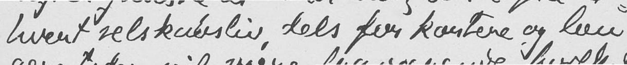hvert selskabsliv, dels for kortere og læn-
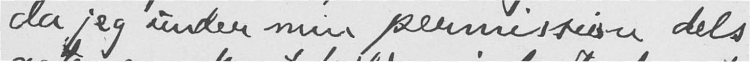da jeg under min permission dels
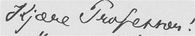Kjære Professor!
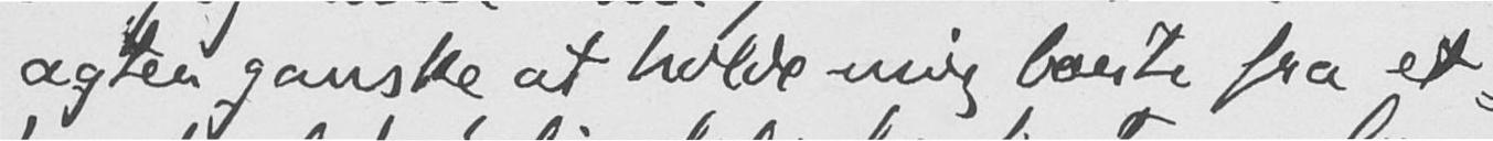agter ganske at holde mig borte fra et-
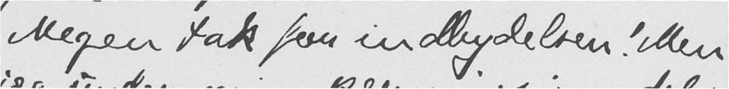Megen tak for indbydelsen! Men
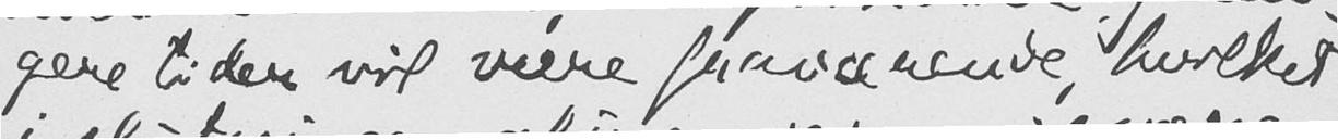gere tider vil være fraværende, hvilket
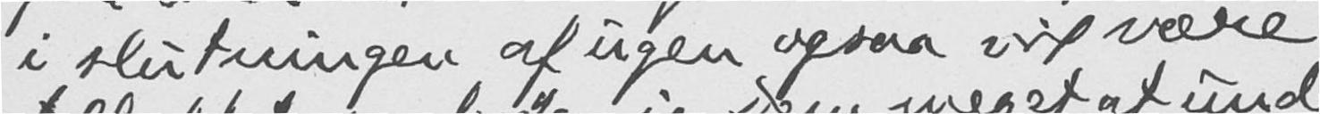i slutningen af ugen ogsaa vil være
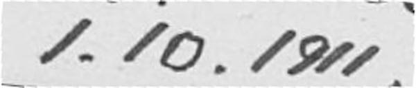1. 10. 1911.
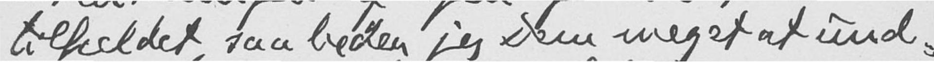tilfældet, saa beder jeg Dem meget at und-
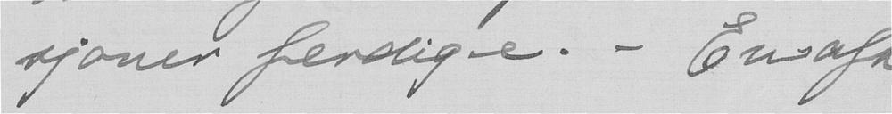sjoner ferdige. - En aften
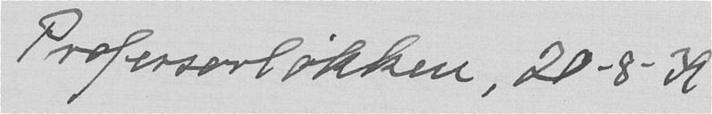Professerløkken, 20-8-39
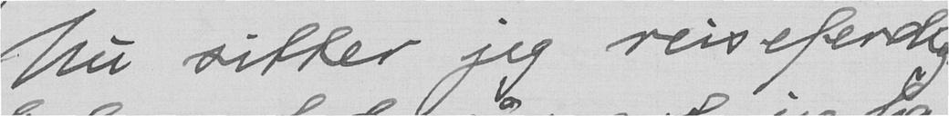Nu sitter jeg reiseferdig
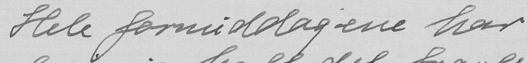Hele formiddagene har
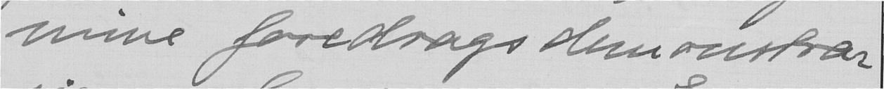mine foredragsdemonstra-
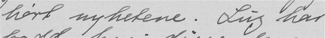hørt nyhetene. Luz har
